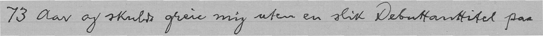73 Aar og skulde greie mig uten en slik Debuttanttitel paa
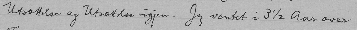Utsættelse og Utsættelse igjen. Jeg ventet i 3½ Aar over
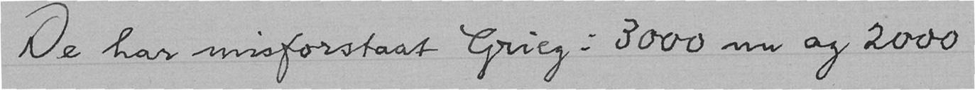De har misforstaat Grieg: 3000 nu og 2000
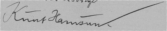Knut Hamsun.
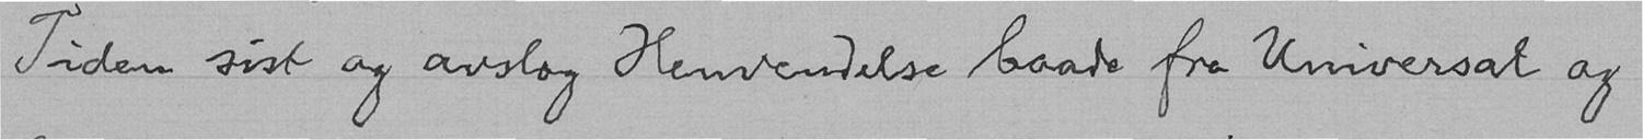Tiden sist og avslog Henvendelse baade fra Universal og
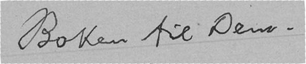Boken til Dem.
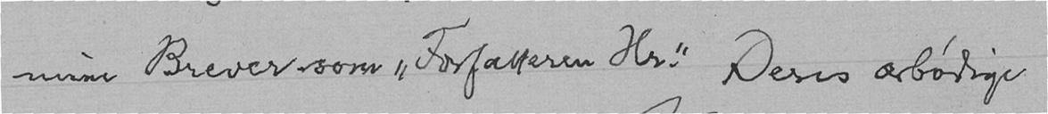mine Brever som "Forfatteren Hr." Deres ærbødige
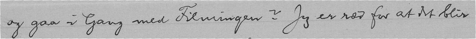og gaa i Gang med Filmingen? Jeg er ræd for at det blir
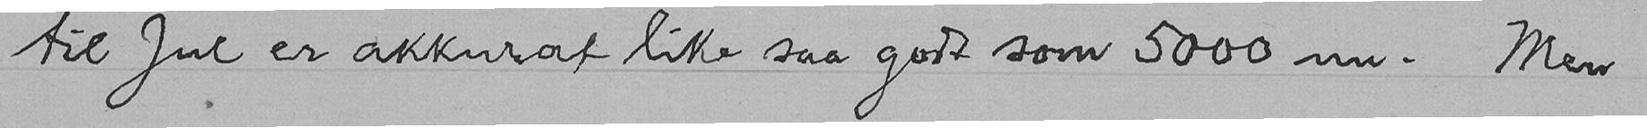til Jul er akkurat like saa godt som 5000 nu. Men
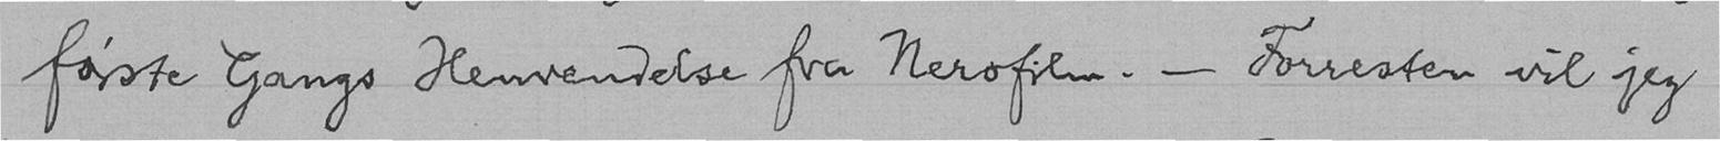første Gangs Henvendelse fra Nerofilm. - Forresten vil jeg
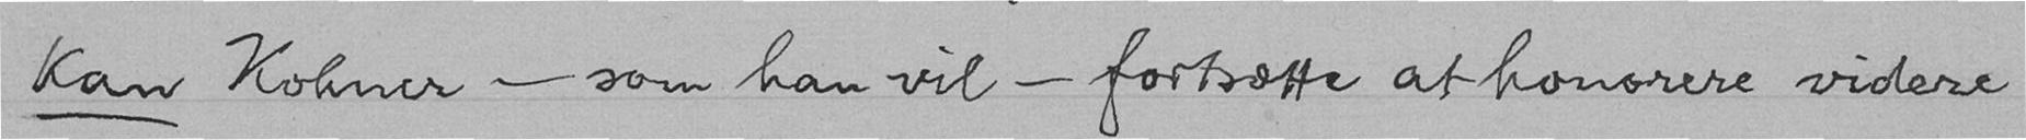kan Kohner - som han vil - fortsætte at honorere videre
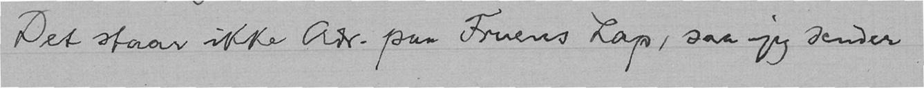Det staar ikke Adr. paa Fruens Lap, saa jeg sender
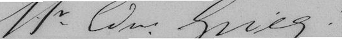Mr Edv. Grieg!
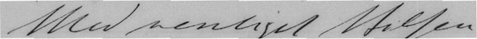Med venligst Hilsen
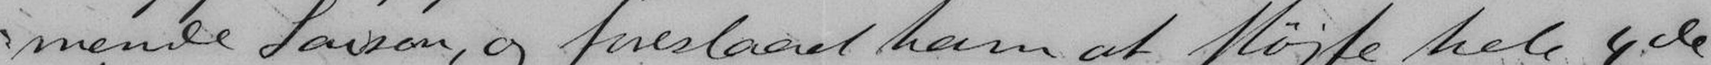mende Saison, og foreslaaet ham at sløyfe hele 4de
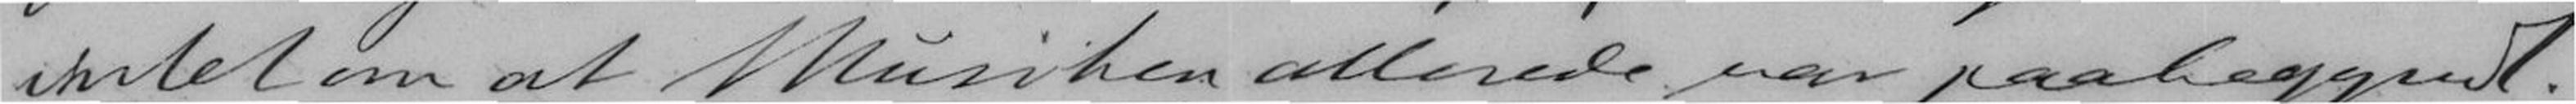intet om at Musiken allerede var paabegyndt.
